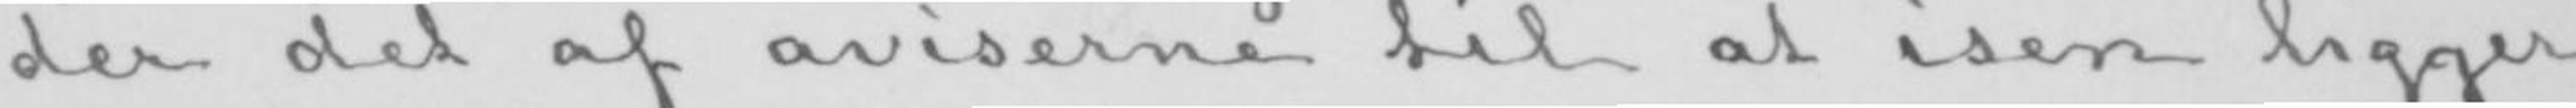der det af aviserne til at isen ligger
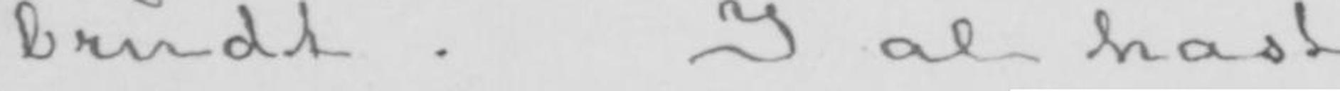brudt. I al hast
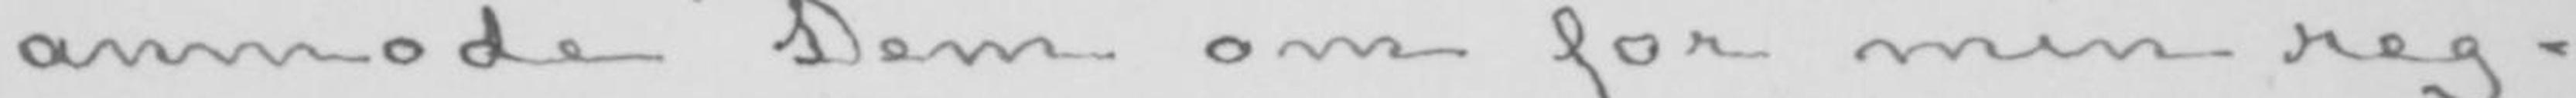anmode Dem om for min reg-
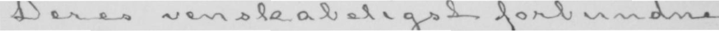Deres venskabeligst forbundne
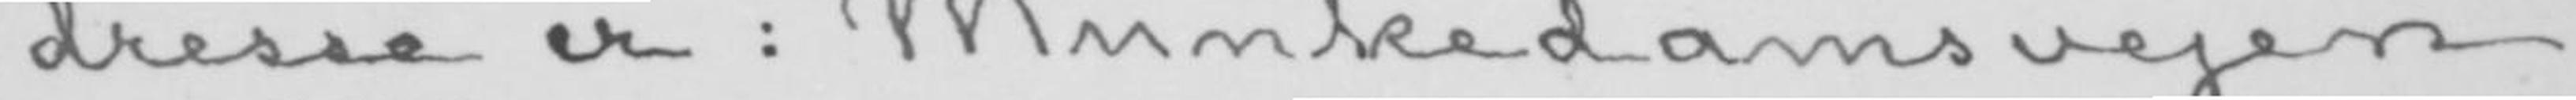dresse er: Munkedamsvejen,
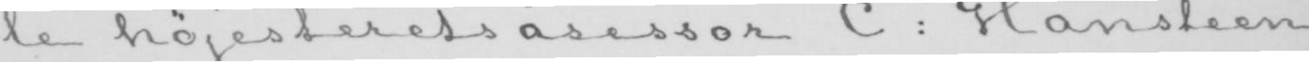le højesteretsasessor C: Hansteen
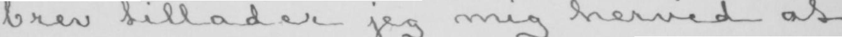brev tillader jeg mig herved at
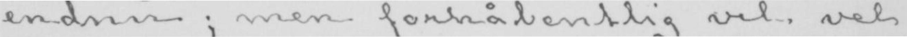endnu; men forhåbentlig vil vel
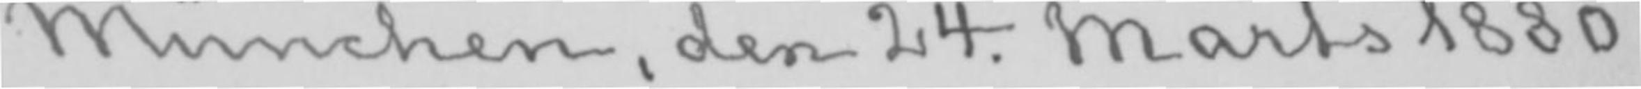München, den 24. Marts 1880.
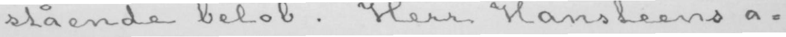stående beløb. Herr Hansteens a-
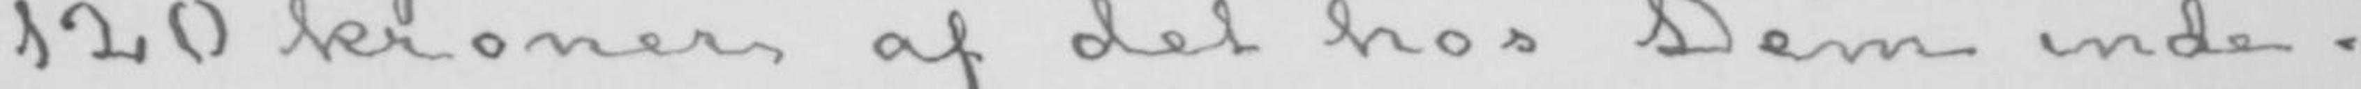120 kroner af det hos Dem inde-
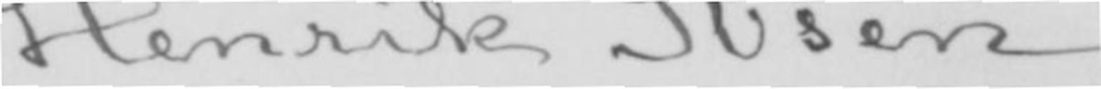Henrik Ibsen.
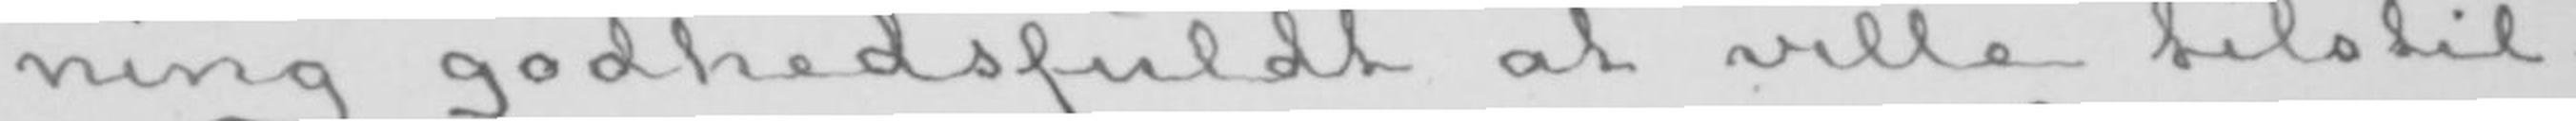ning godhedsfuldt at ville tilstil-
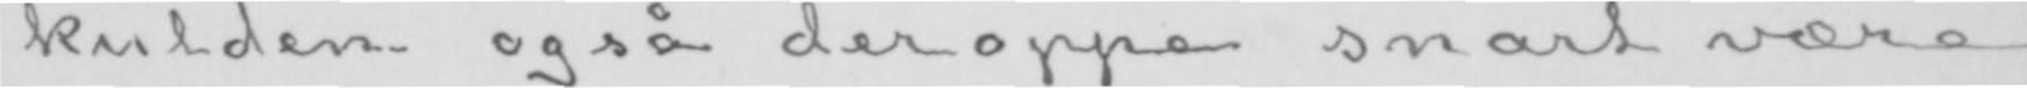kulden også deroppe snart være
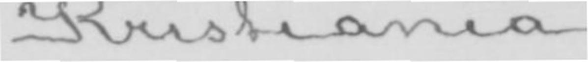Kristiania.
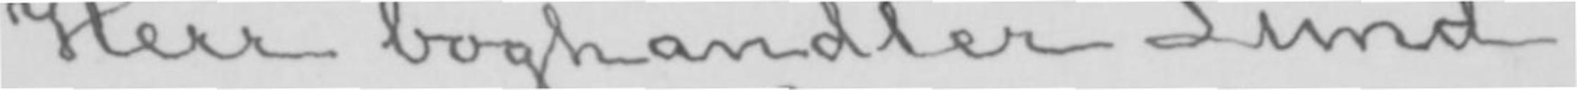Herr boghandler Lund.
